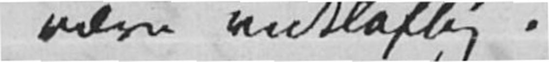være vidtløftig.
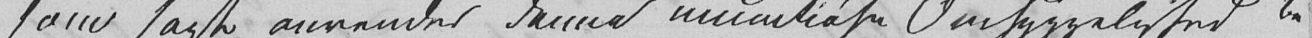som sagt anvender denne minutiøse Omhyggelighed
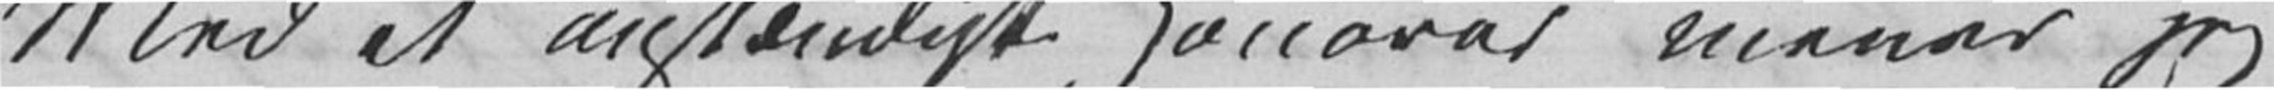Med et anstændigt Honorar mener jeg
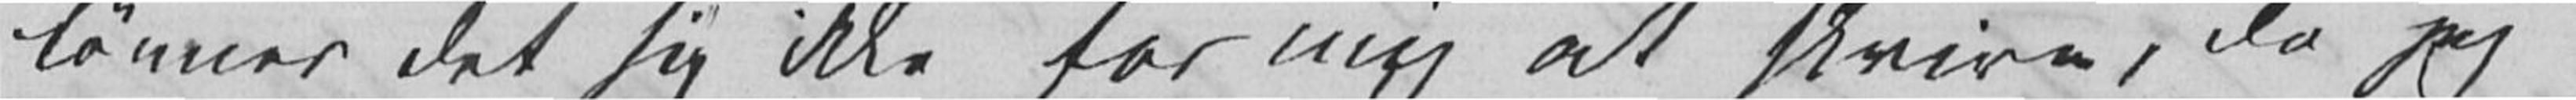lønner det sig ikke for mig at skrive, da jeg
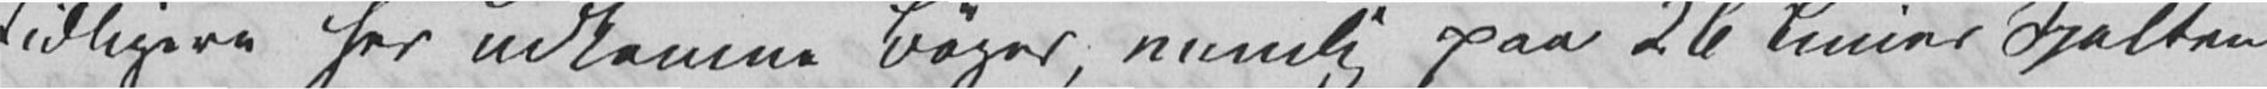tidligere her udkomne Bøger, nemlig paa 26 Linier Spalten
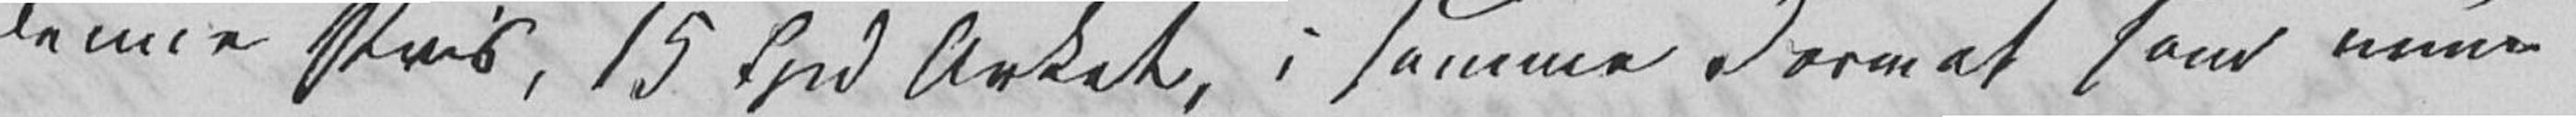denne Pris, 15 Spd Arket, i samme Format som mine
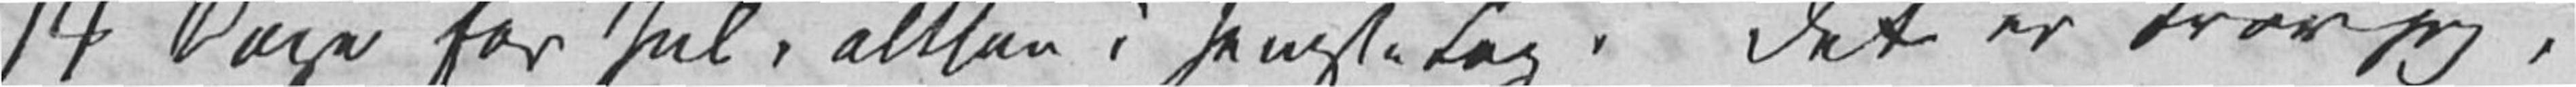14 Dage før Jul, altsaa i seneste Lag. Det er tror jeg,
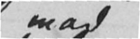mod
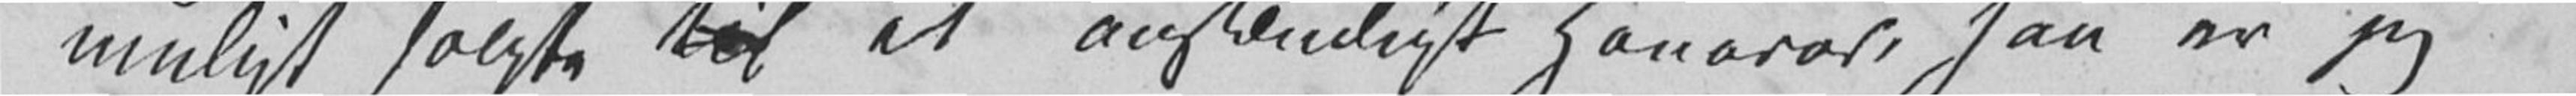muligt solgt til et anstændigt Honorar, saa er jeg
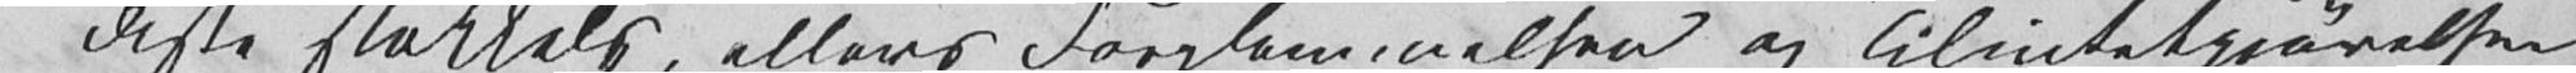disse stakkels, ellers Forglemmelsen og Tilintetgjørelsen
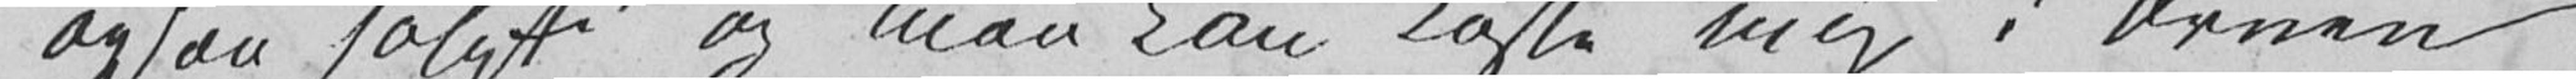ogsaa «solgt» og man kan kaste mig i Ovnen
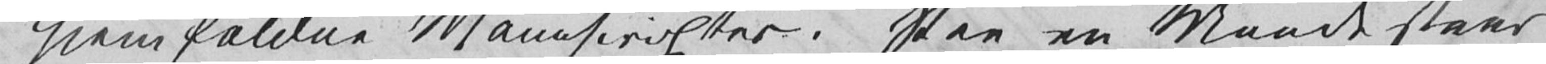hjemfaldne Manuscripter. Paa en Maade staar
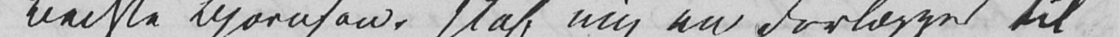Bedste Bjørnson! Skaf mig en Forlægger til
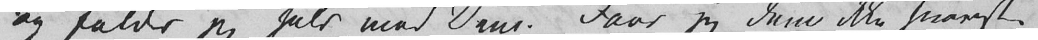og falder jeg selv med Dem. Faar jeg dem ikke snarest
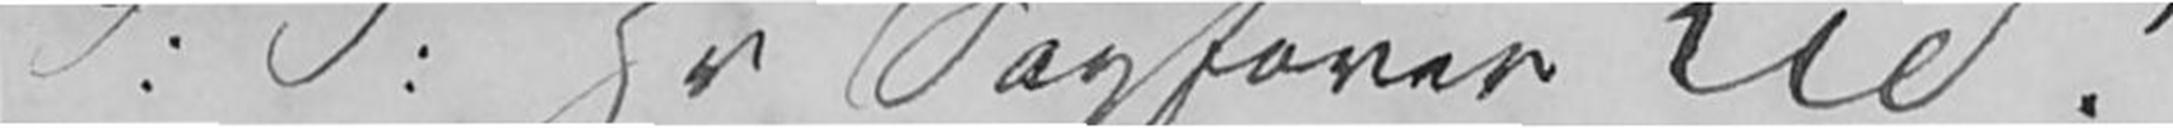S: T: Hr Sagfører Lie!
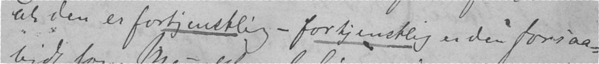at den er fortjenstlig – fortjenstlig er den forsaa-
